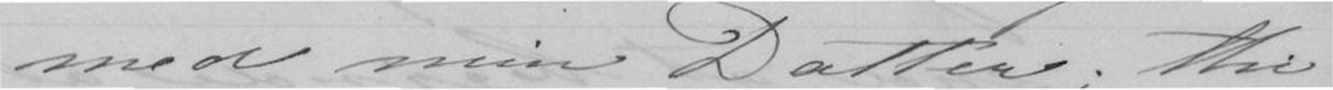med min Datter; Thi
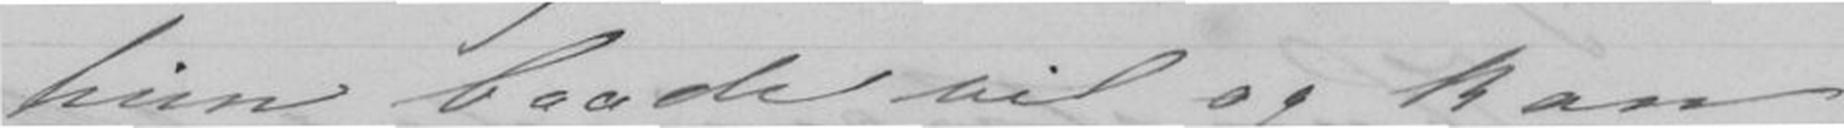hun baade vil og kan
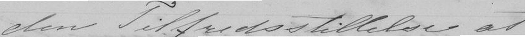den Tilfredsstillelse at
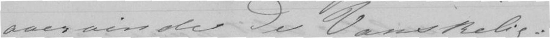overvinde de Vanskelig-
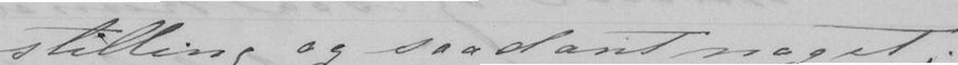stilling og saadant noget;
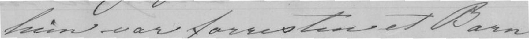hun var forresten et Barn
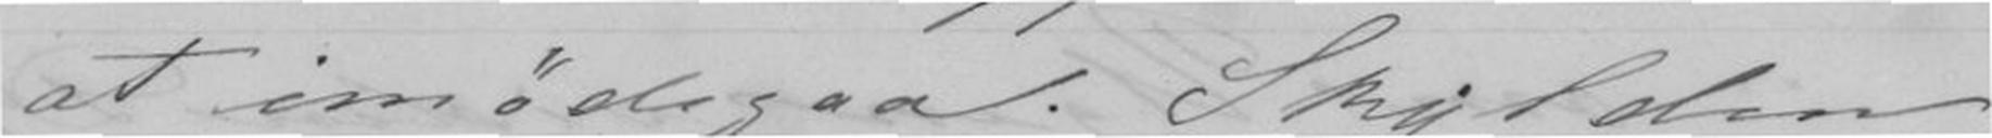at imødegaa. Skylden
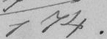7/1.74.
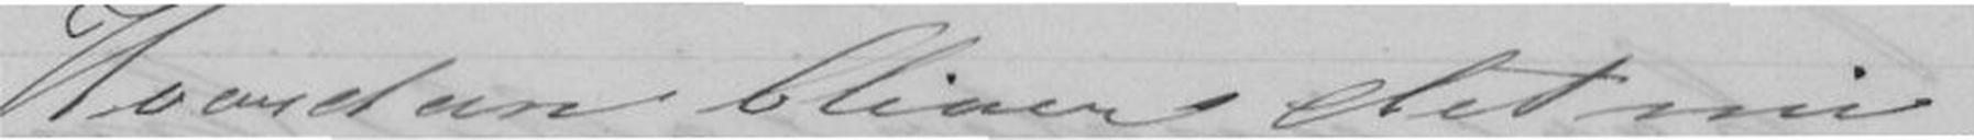Hvordan bliver det nu,
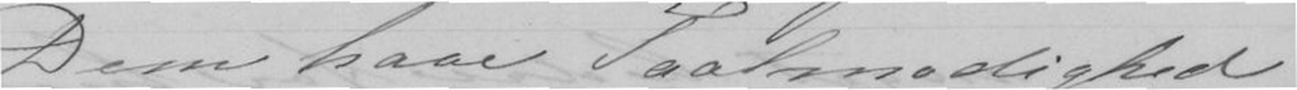Dem have Taalmodighed
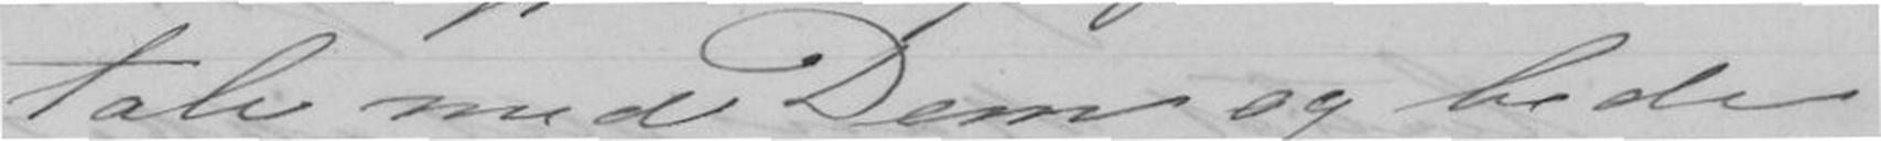tale med Dem og bede
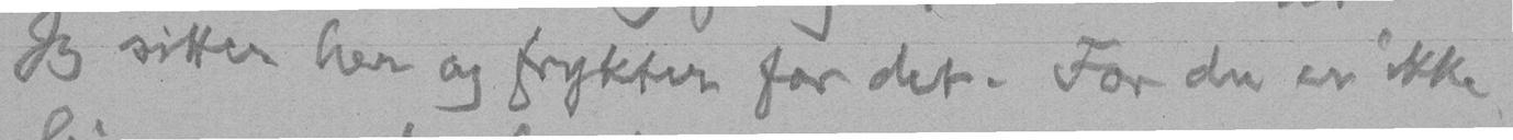Jeg sitter her og frykter for det. For du er ikke
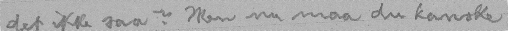det ikke saa? Men nu maa du kanske
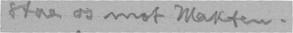staa os mot Makten.
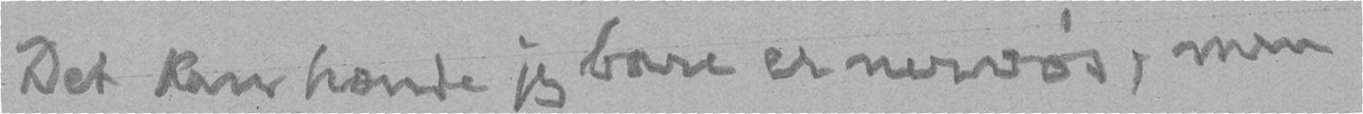Det han hænde jeg bare er nervøs, men
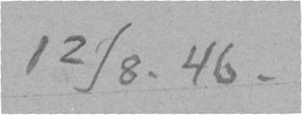12/8. 46.
